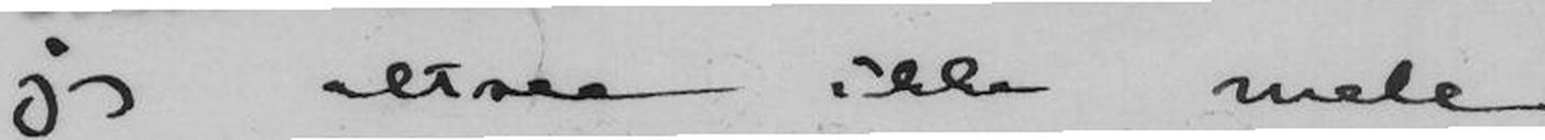jeg altsaa ikke mele
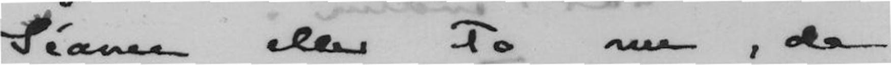Séance eller to nu, da
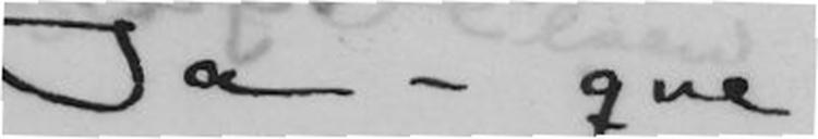Ja - que
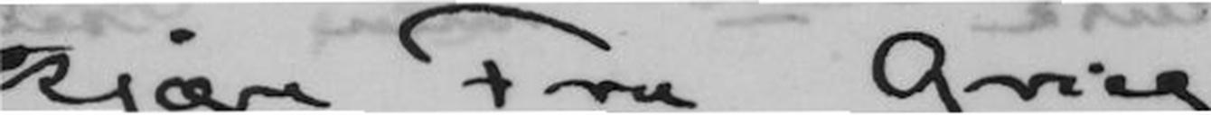Kjære Fru Grieg
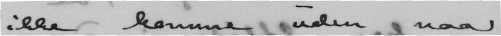ikke komme uden naar
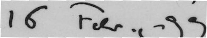16 Febr. - 99
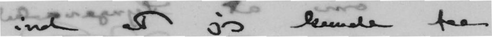ind at jeg kunde faa
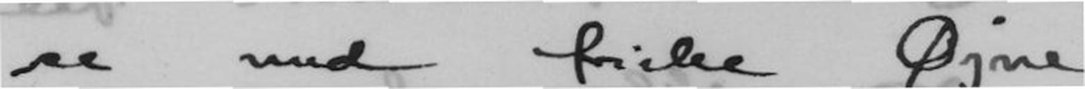se med friske Øjne
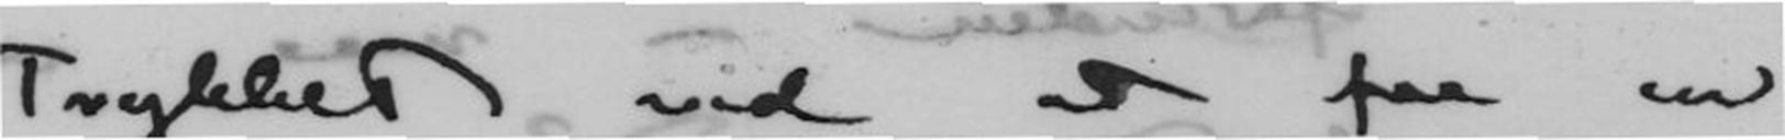trykket ved at faa en
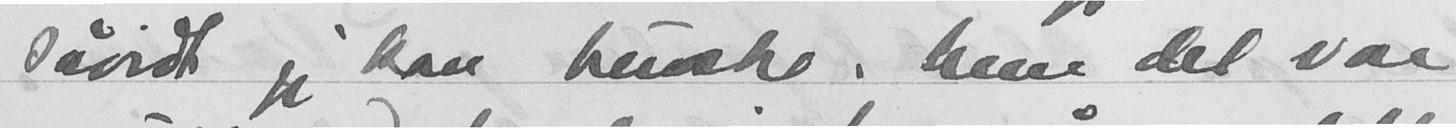såvidt jeg kan huske, men det var
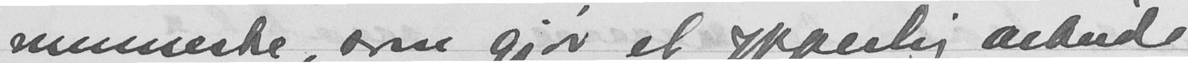menneske, som gjør et ypperlig arbeide
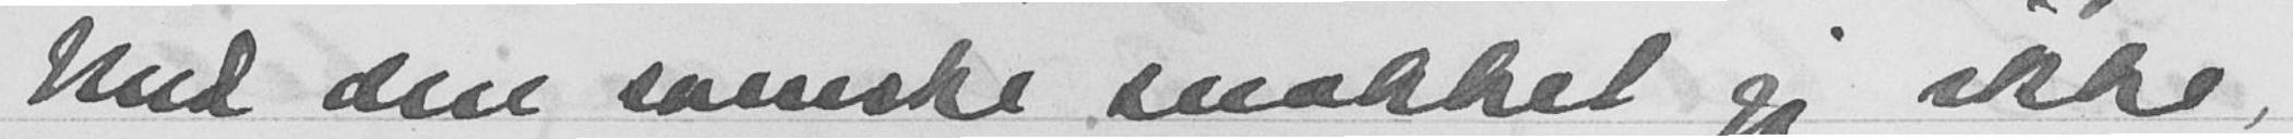Med den svenske snakket jeg ikke,
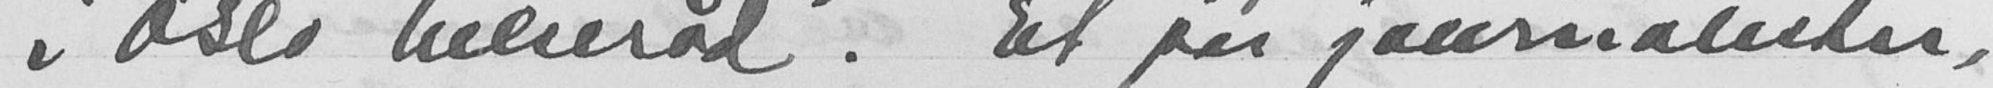i Oslo helseråd. Et par journalister,
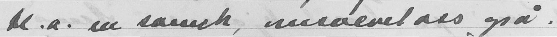bl.a. en svensk, omsvevet oss også.
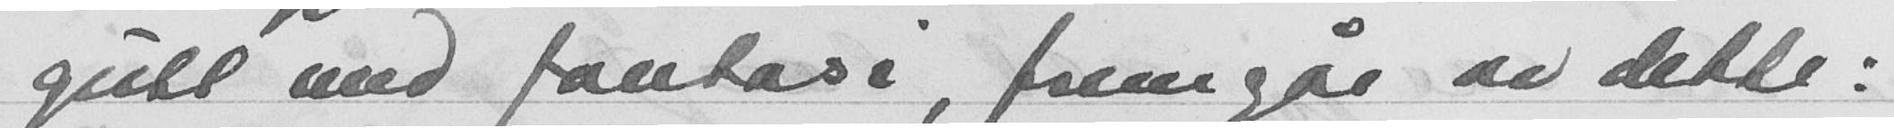gutt med fantasi, fremgår av dette:
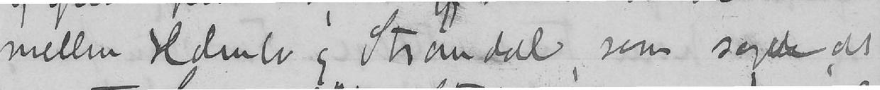mellem Holmbo og Strømdal, som sagde, at
